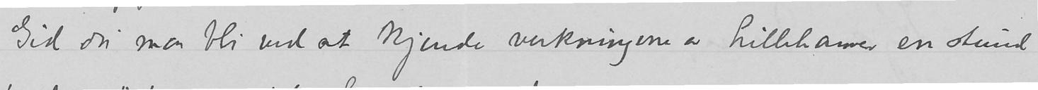Gid du maa bli ved at kjende virkningene av Lillehamer en stund
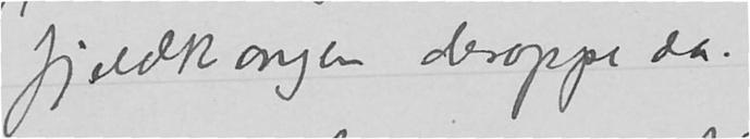fjeldkongen deroppe da.
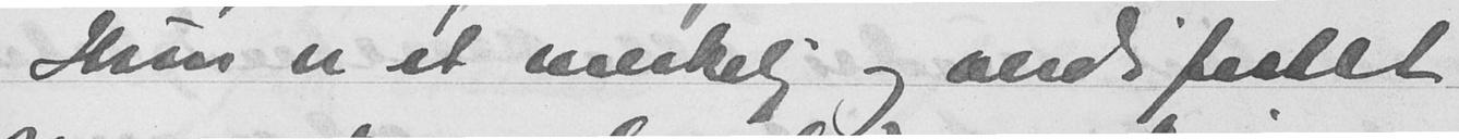Han er et merkelig og åndsfullt
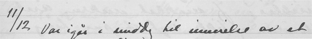11/12 Var igår i middag til innvielse av et
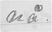nå.
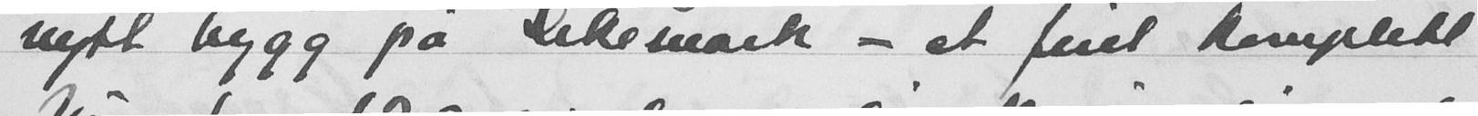nytt bygg pa Lilomark - et fint komplett
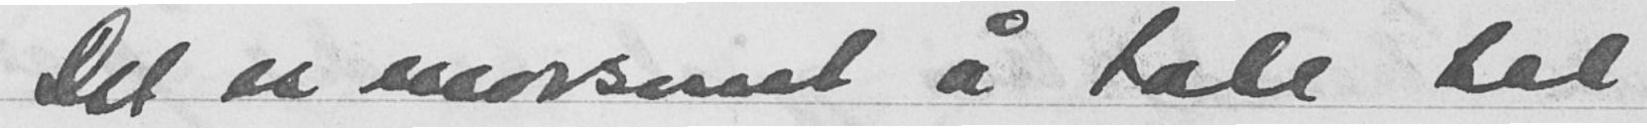Det er morsomt å tale til
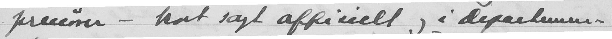precorer - kort sagt officielt og i departemen-
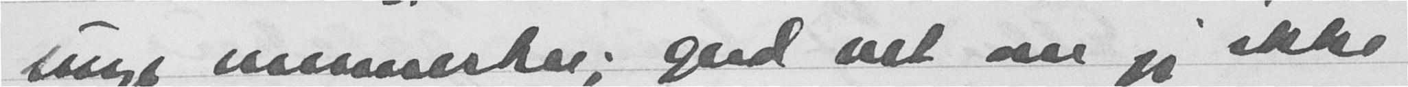unge mennesker; gud vet om jeg ikke
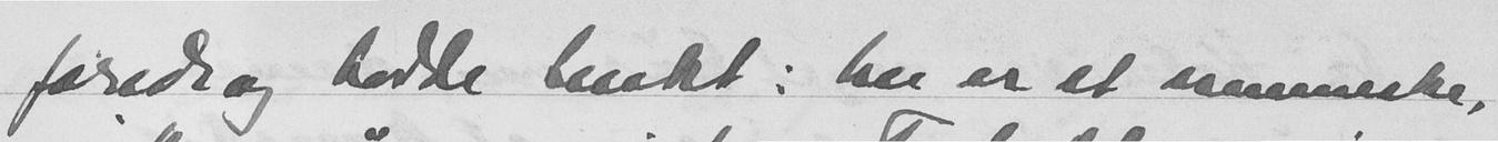foredrag hadde tenkt: her er et menneske,
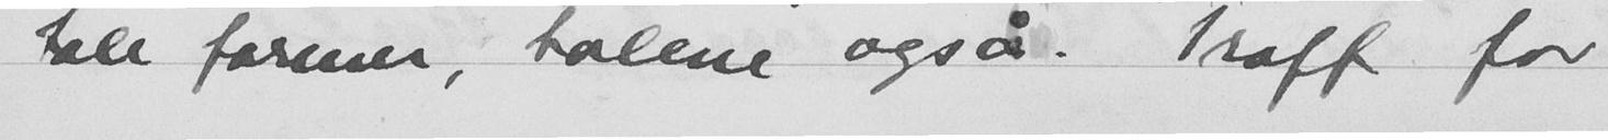tale former, talene også. Traff for
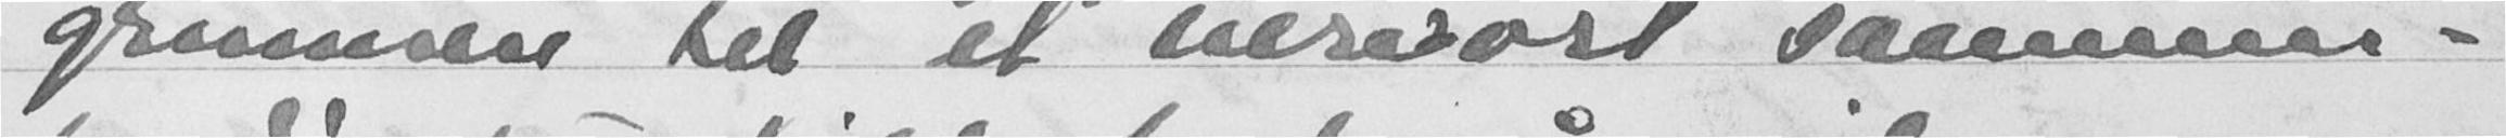grunnen til et nervøst sammen-
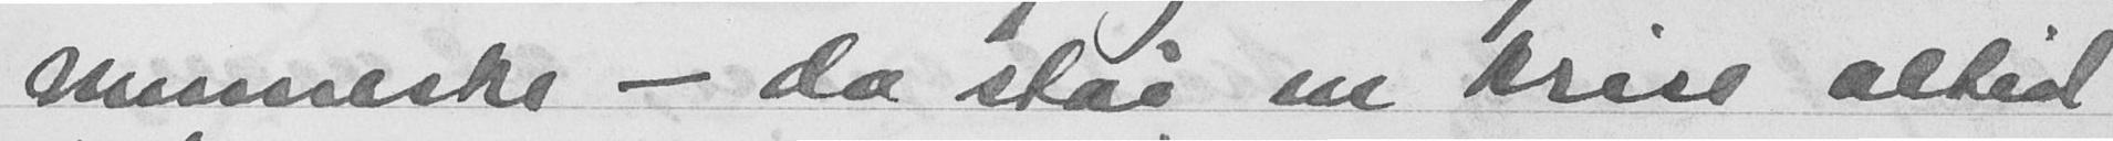menneske - da står en krise altid
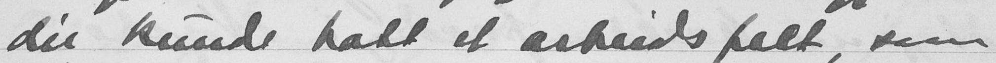dér kunde hatt et arbeidsfelt, som
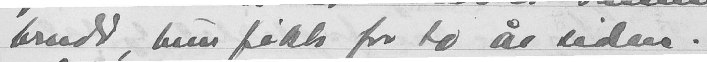brudd, hun fikk for to år siden.
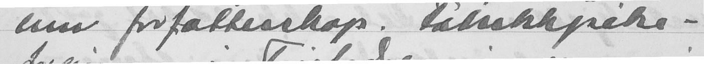enn forfatterskap. Fabrikkpike-
